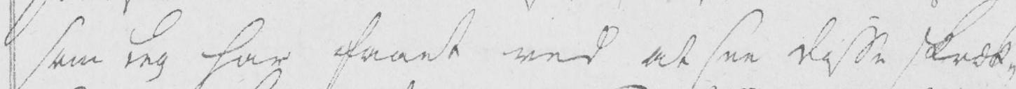som jeg har faaet ved at see disse skræk-
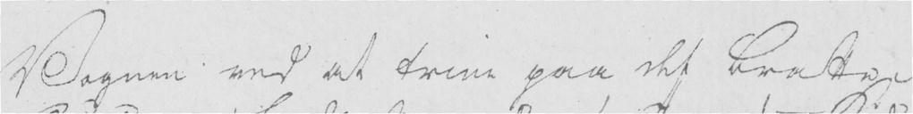Vognen ved at trine paa det bratte
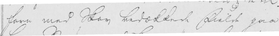forn med Skov bedækkede Fielde paa
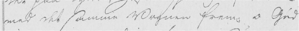med det samme Vognen frem; o Gud
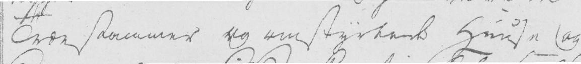Træestammer og omstyrtede Huuse og
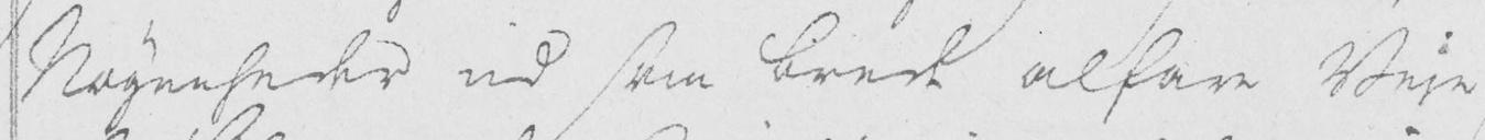Nøgenheder ud som brede alfare Veje
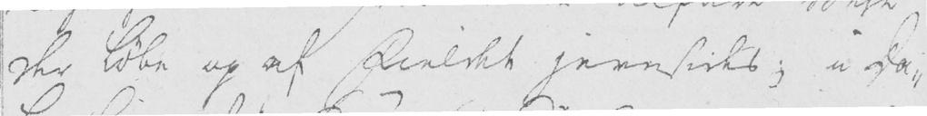der løbe op af Fieldet jevnsides; i Da-
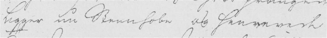ligger nu Steenhobe og henrevede
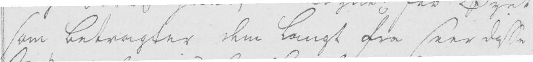som betragter dem langt fra seer disse
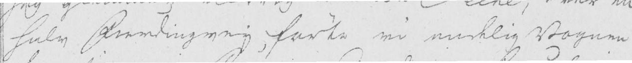halv Fierdingvey førte vi endelig Vognen
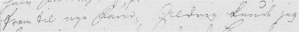frem til nye Farer. Aldrig kunde jeg
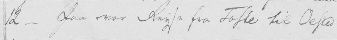12 - Paa vor Reyse fra Tofte til Olstad
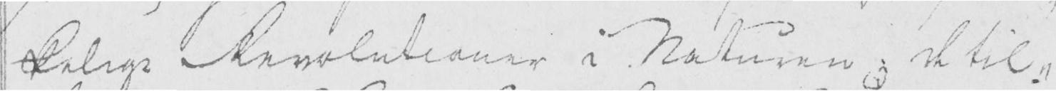kelige Revolutioner i Naturen; de til-
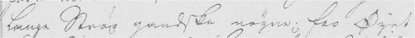lange Strax gandske nøgne; for Øyet
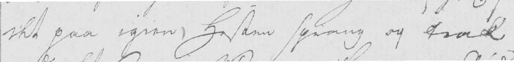det paa igien, Hesten sprang og trakk
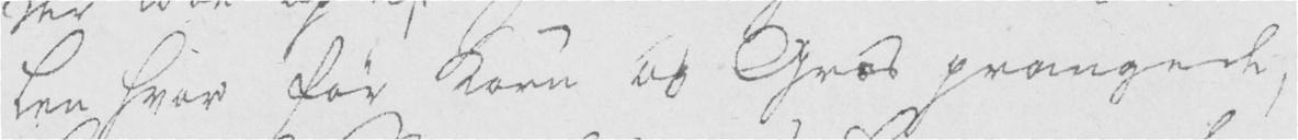len hvor før Korn og Græs prangede,
